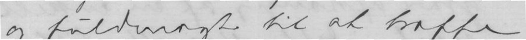og fuldmagt til at træffe
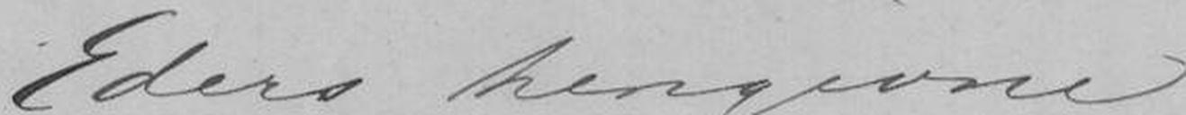Eders hengivne
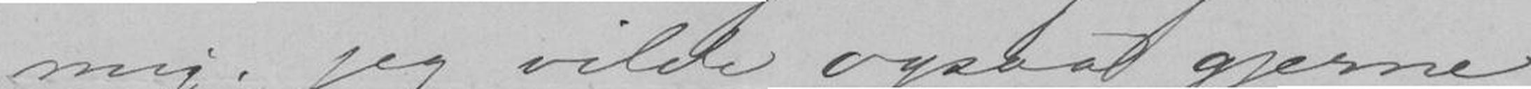mig; jeg vilde ogsaa gjerne
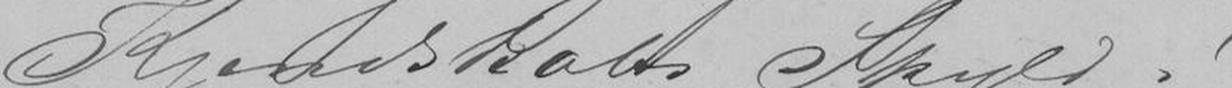Kjendskabs Skyld!
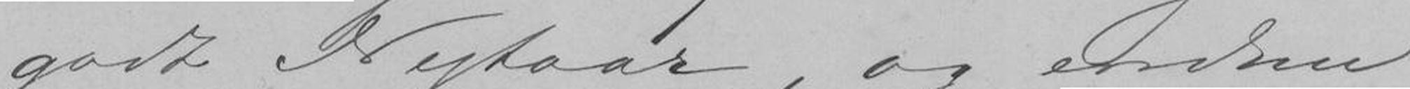godt Nytaar, og endnu
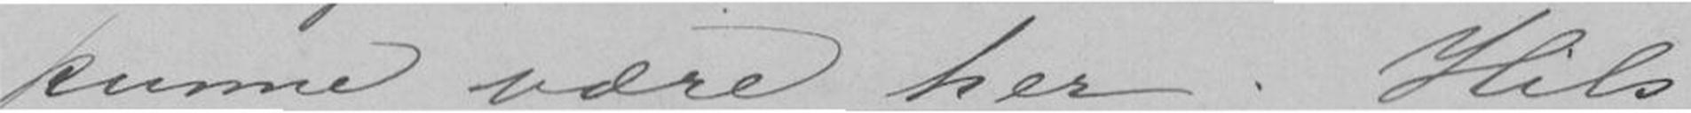kunne være her. Hils
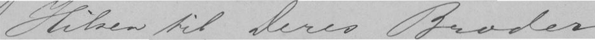Hilsen til deres Broder
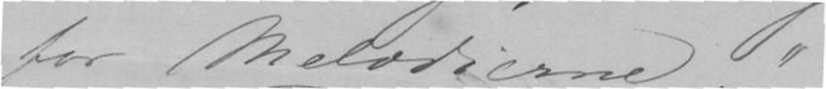for Melodierne.
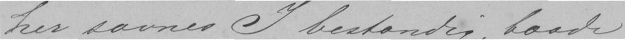her savnes I bestandig; baade
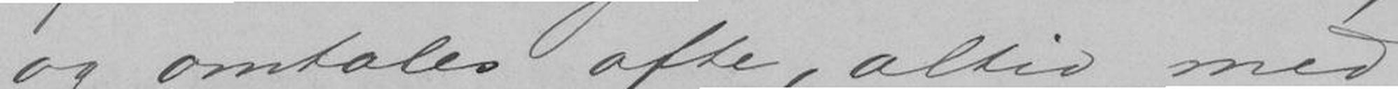og omtales ofte, altid med
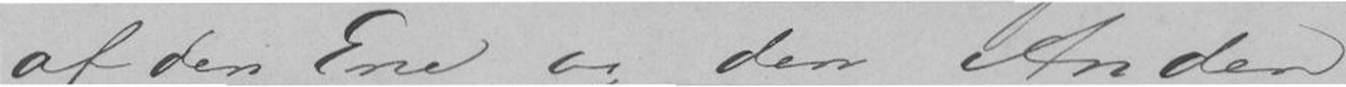af den Ene og den Anden,
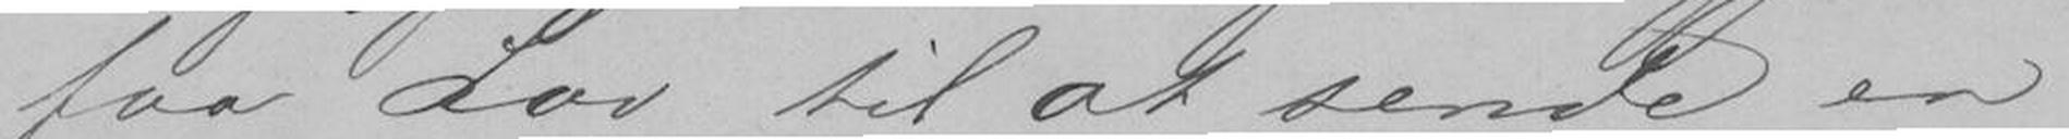faa Lov til at sende en
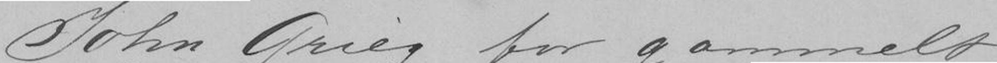John Grieg for gammelt
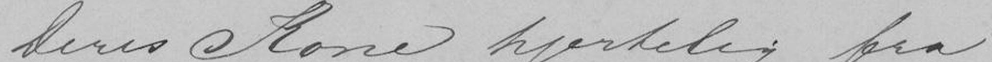Deres Kone hjertelig fra
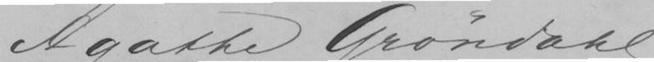Agathe Grøndahl
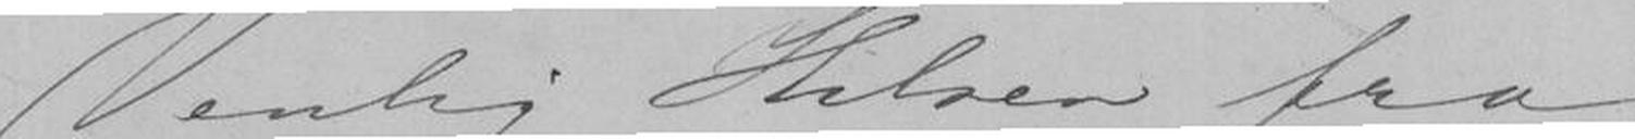Venlig Hilsen fra
